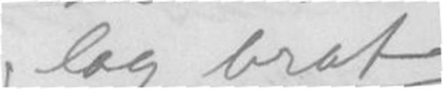lag brat,
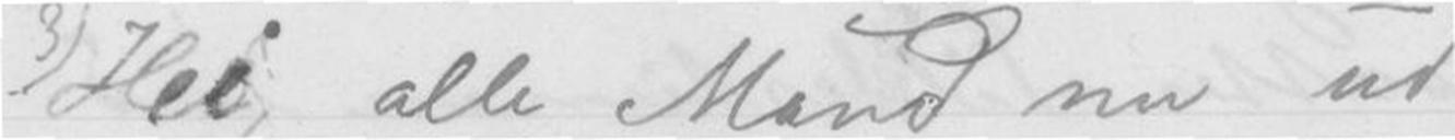3) Hei alle Mand nu ud
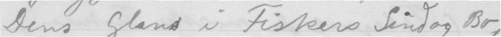Dens Glands i Fiskers Sind og Bo,
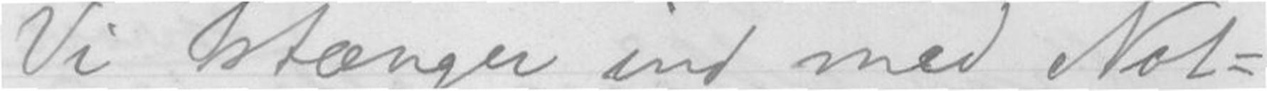Vi stænger ind med Not-
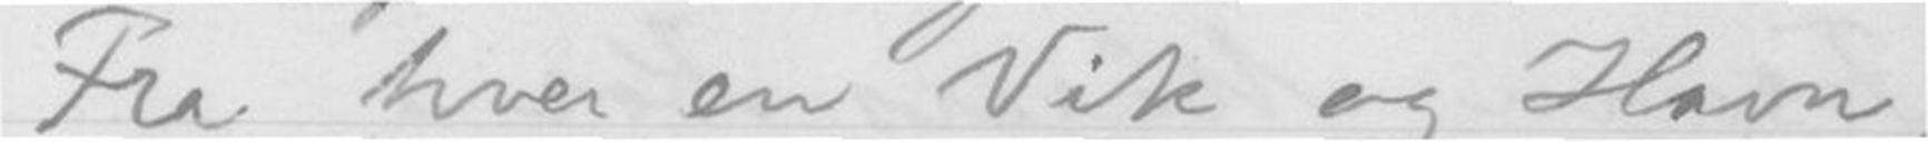Fra hver en Vik og Havn,
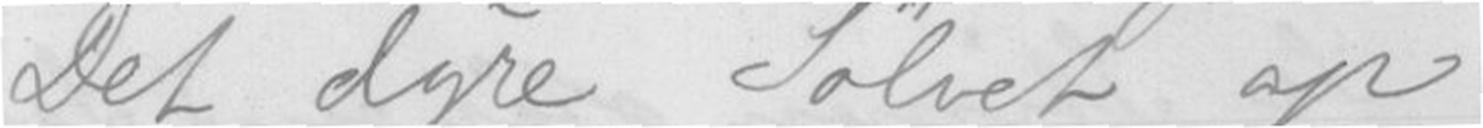Det Dyre Sølvet op
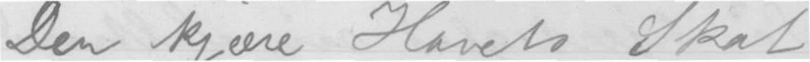Den kjære Havets Skat
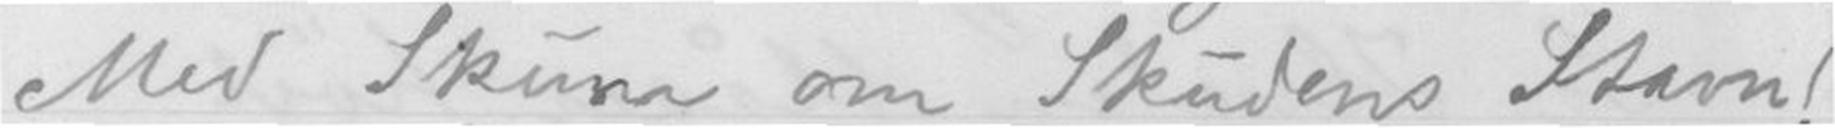Med Skum om Skudens Stavn!
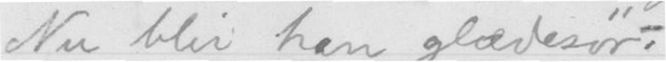Nu blir han gædesør
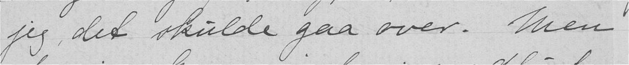jeg det skulde gaa over. Men
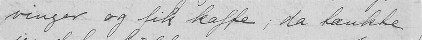vinger og fik kaffe; da tænkte
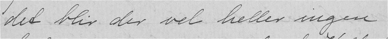det blir der vel heller ingen
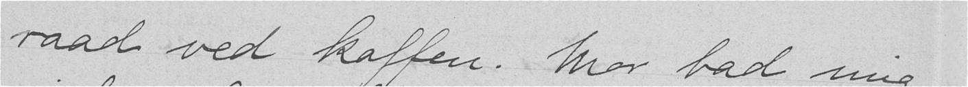raad ved kaffen. Mor bad mig
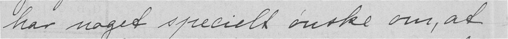har noget specielt ønske om, at
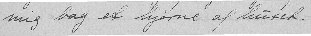mig bag et hjørne af huset.
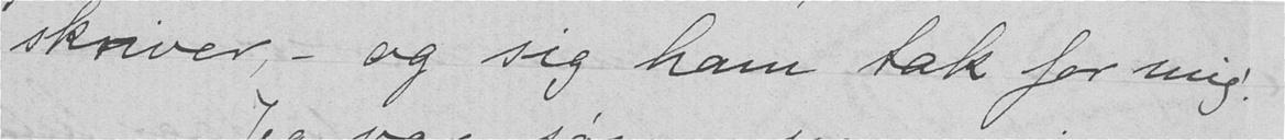skriver,- og sig ham tak for mig!
